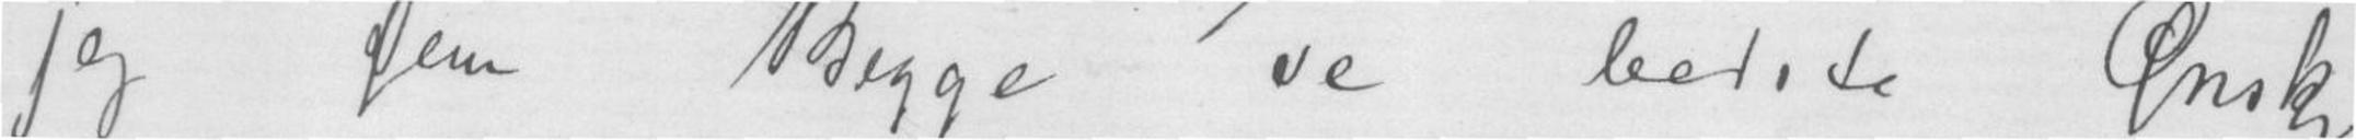jeg Dem Begge De bedste ønsker
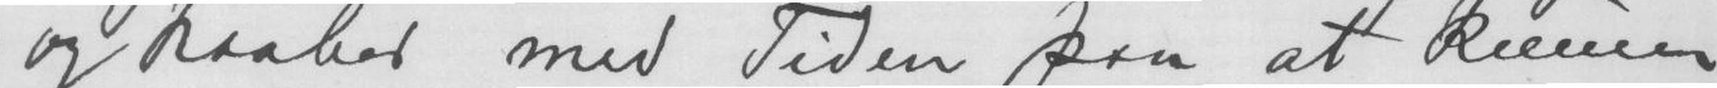og haaber med Tiden paa at kunne
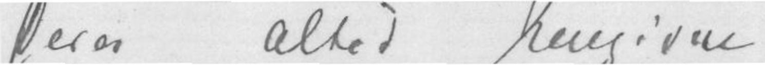Deres altid hengivne
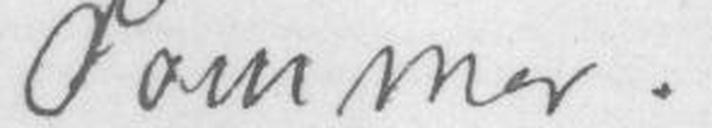Sommer.
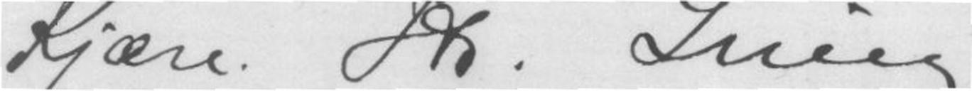Kjære Hr. Grieg!
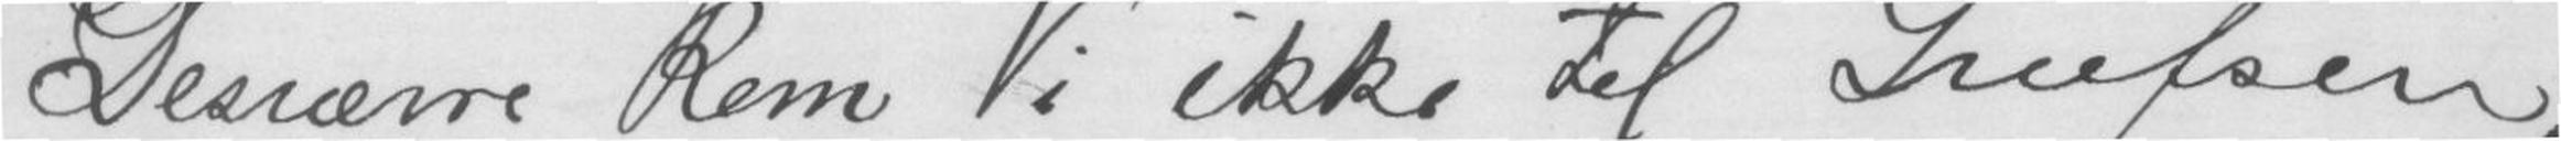Desværre kom Vi ikke til Gufsen,
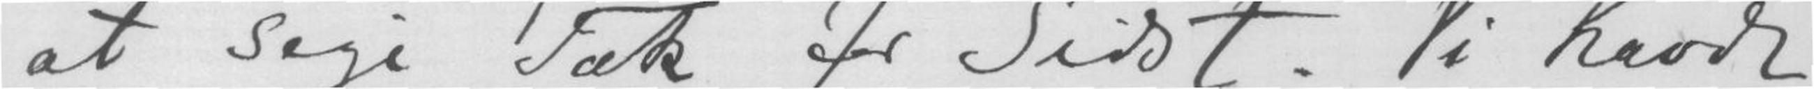at sige Tak for Sidst. Vi havde
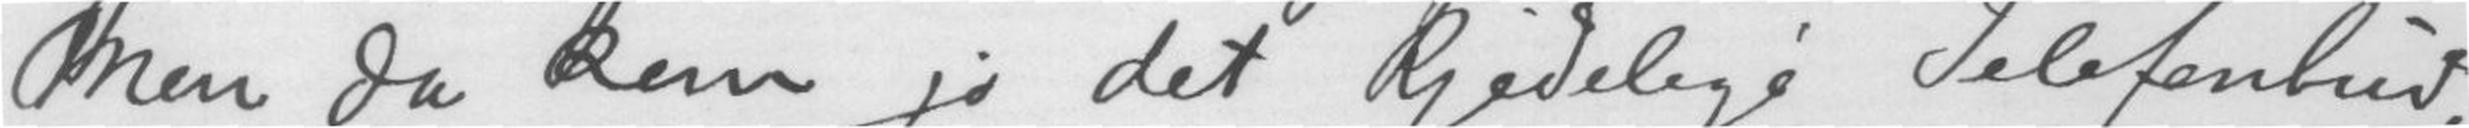men da kom jo det kjedelige telefonbud
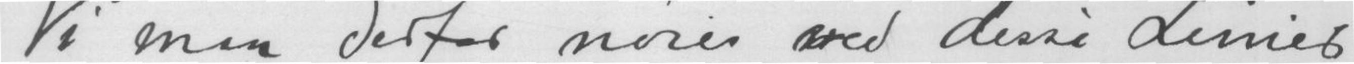Vi maa Derfor nøies med disse Linier
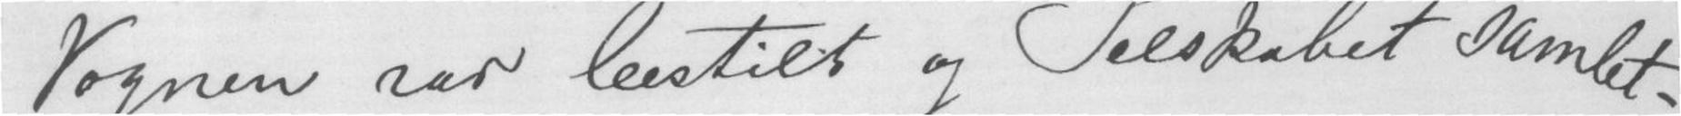Vognen var bestilt og Selskabet samlet-
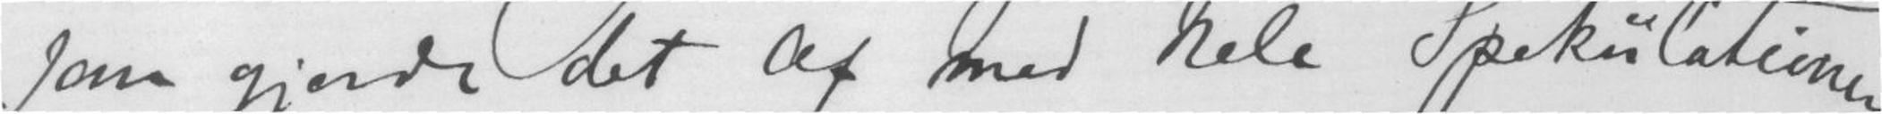som gjorde det af med hele Spekulationen
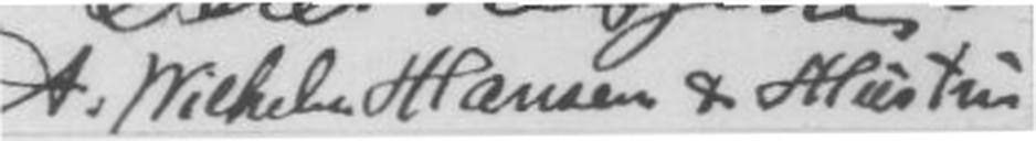A.Mickelsen Hansen & Hustru
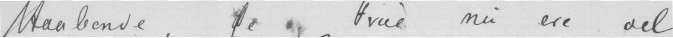Haabende, De og Fruen nu ere vel
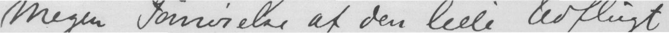meget fornøielse af den lille Udflugt
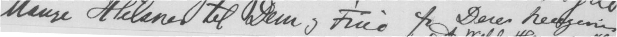Mange Hilsener til Dem og Frue fra Deres hengivne
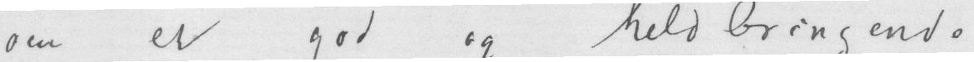om en god og heldbringende
